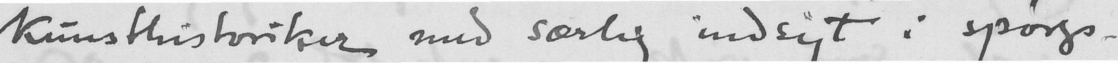kunsthistoriker med særlig indsigt i spørgs-
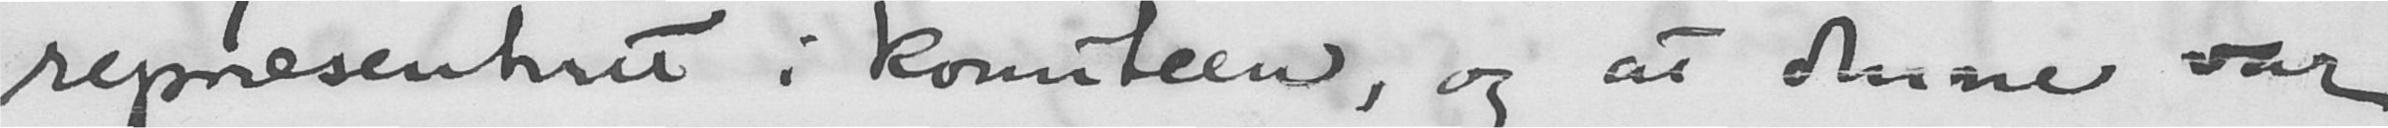representeret i komiteen, og at denne var
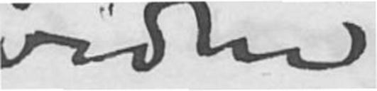viden
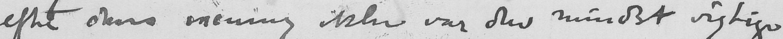efter dens mening ikke var den mindst vigtige
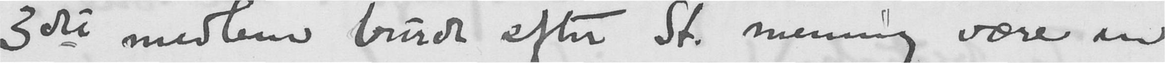3dje medlem burde efter St. mening være en
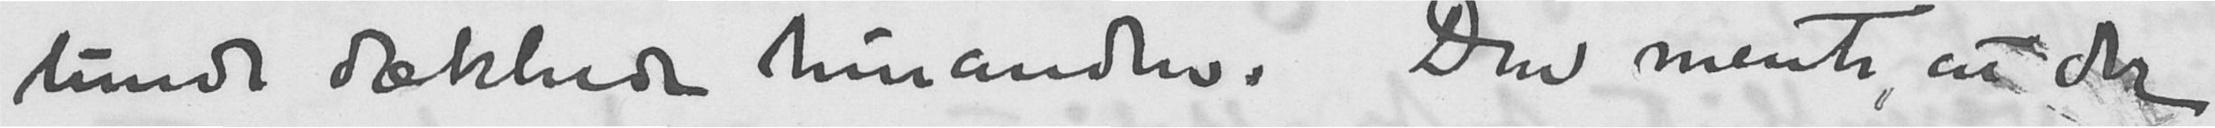lunde dækkede hinanden. Den mente, at der
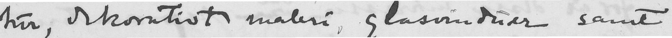tur, dekorativt maleri, glasvinduer samt
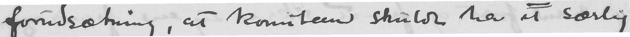forudsætning, at komiteen skulde ha et særlig
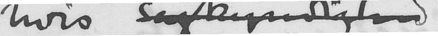hvis sagkyndighed
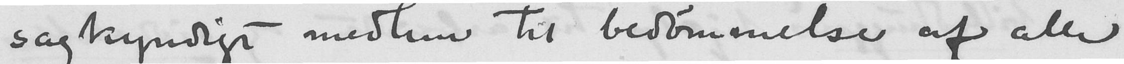sagkyndigt medlem til bedømmelse af alle
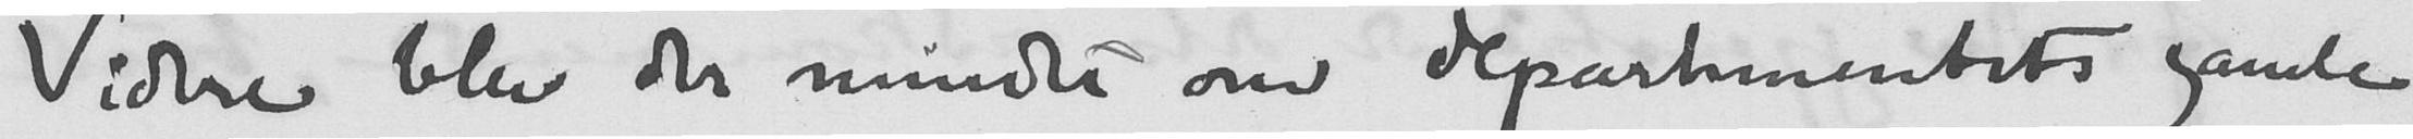Videre blev der mindet om departementets gamle
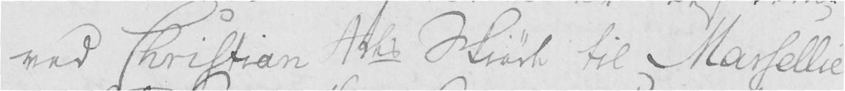ved Christian 4des Skiøde til Marsellie
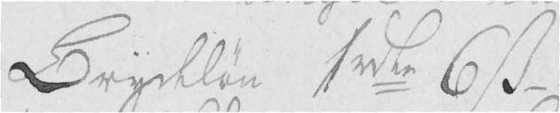Brydløn 1 rdr 6 s -
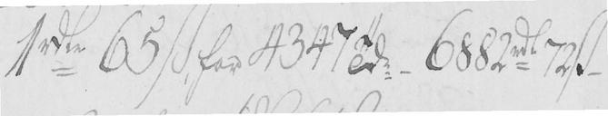1rde 65s for 43472 6882d 7 s
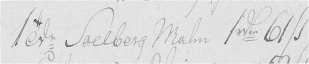1 Tdr Soelberg Malm 1 rde 61 s
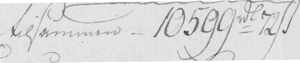tilsammen - 10599 rde 72 s
